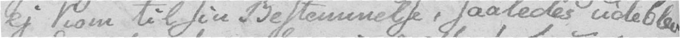ej kom til sin Bestemmelse, saaledes udeblev
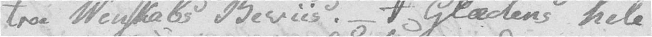troe Venskabs Beviis. – I Glædens hele
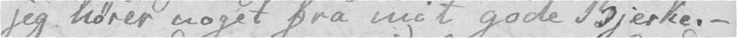jeg hører noget fra mit gode Bjerke. –
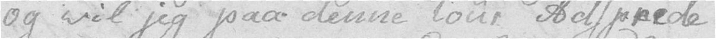og vil jeg paa denne tour Adsprede
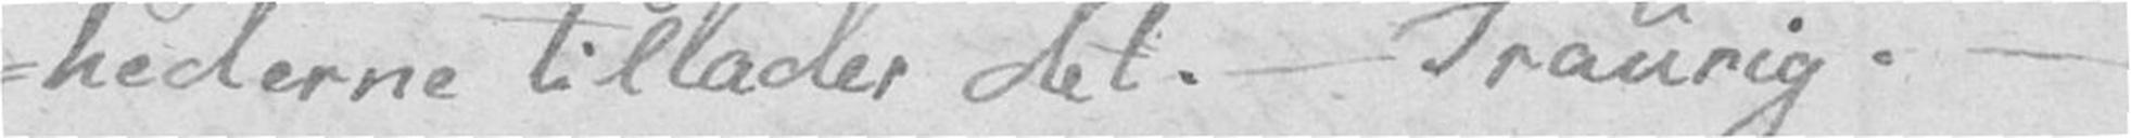-hederne tillader det. – Traurig. –
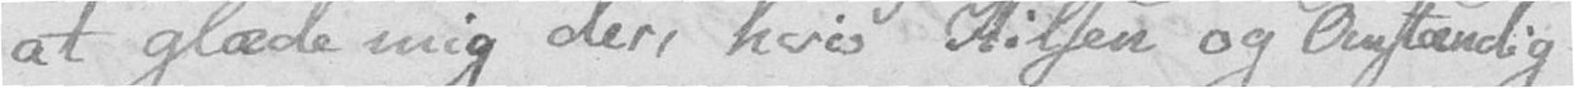at glæde mig der, hvis Hilsen og Omstændig
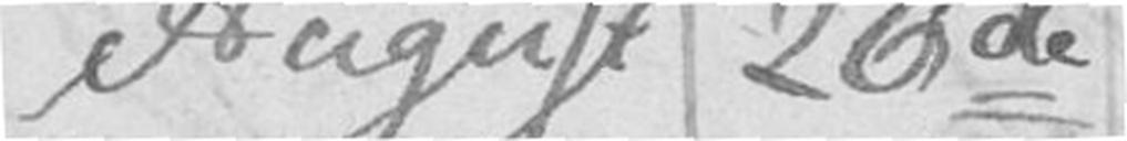August 26de
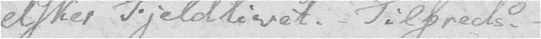elsker Fjeldlivet. Tilfreds. –
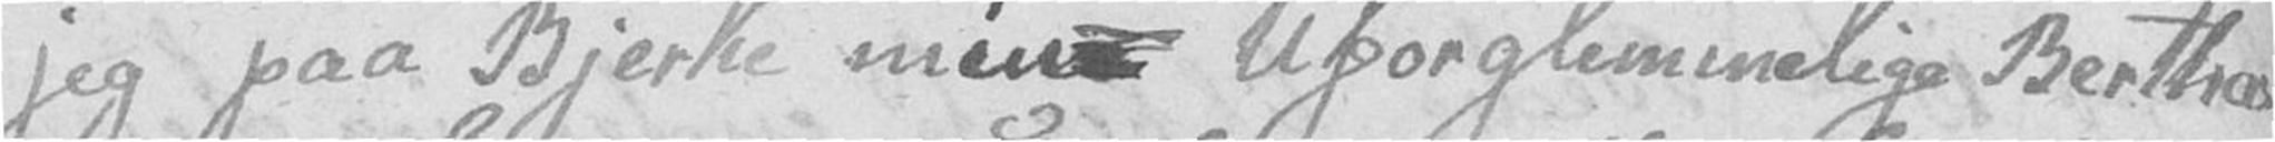jeg paa Bjerke min  uforglemmelige Berthas
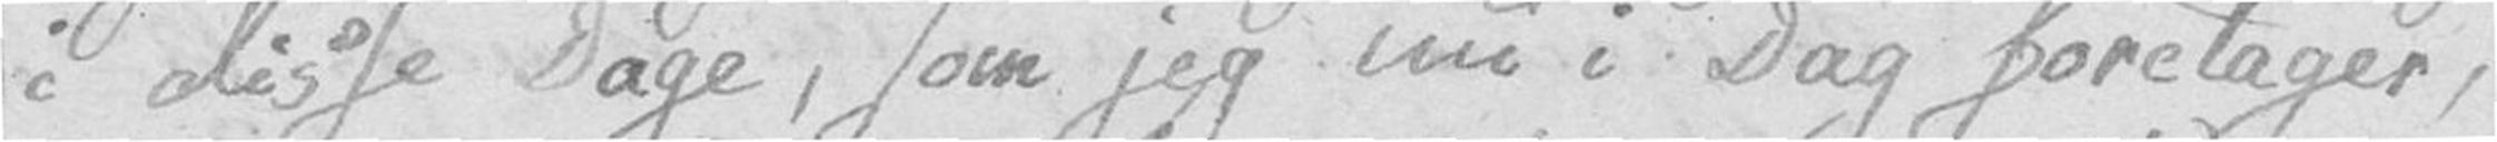i disse Dage, som jeg nu i Dag foretager,
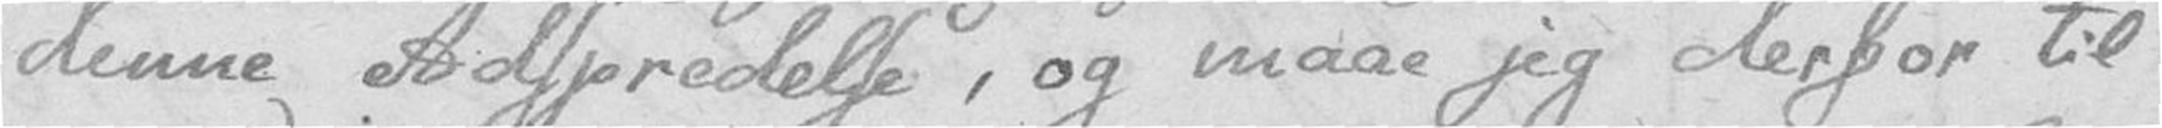denne Adspredelse, og maae jeg derfor til
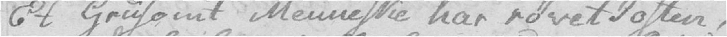Et Grusomt Menneske har røvet Posten,
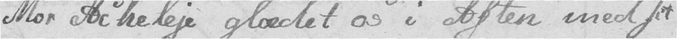Mor Acheleje glædet os i Aften med sit
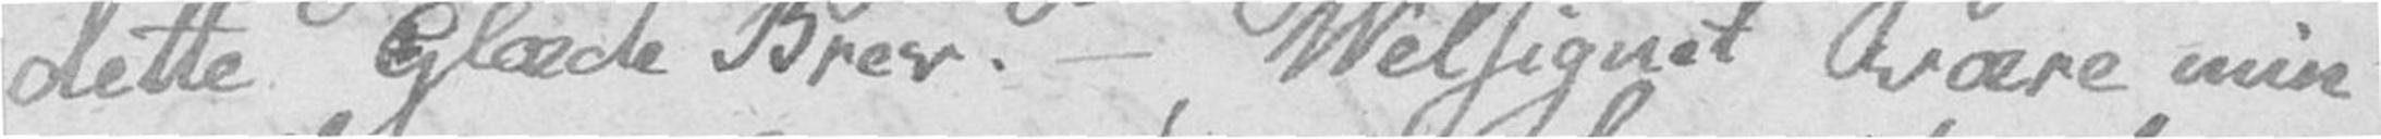dette Glæde Brev. – Velsignet være min
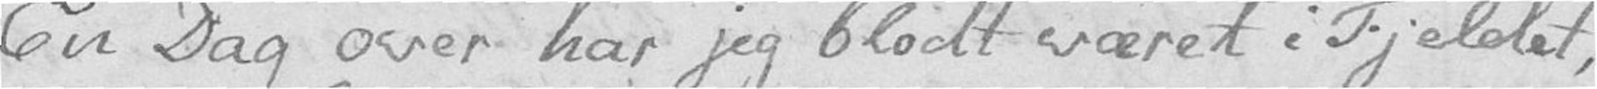En Dag over har jeg blodt været i Fjeldet,
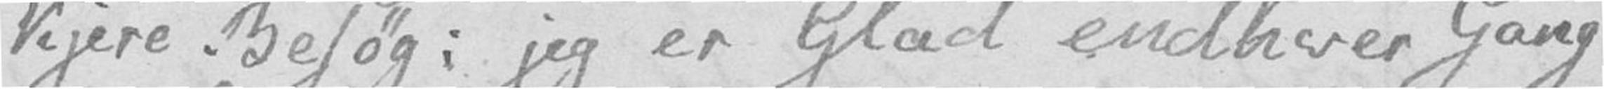kjere Besøg: jeg er Glad endhver Gang
